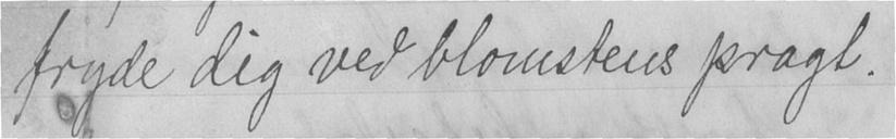fryde dig ved blomstens pragt.
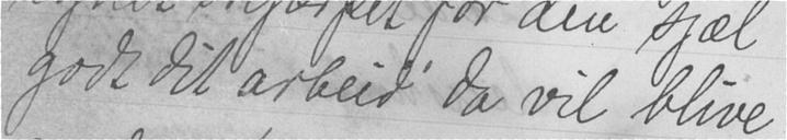godt dit arbeid da vil blive
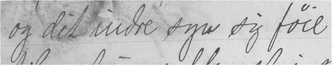og det indre syn sig føie
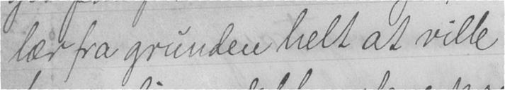lær fra grunden helt at ville
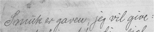Smuk er gaven, jeg vil give:
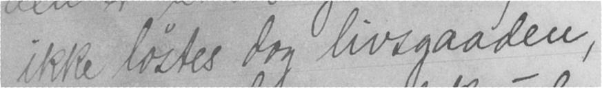ikke løstes dog livsgaaden,
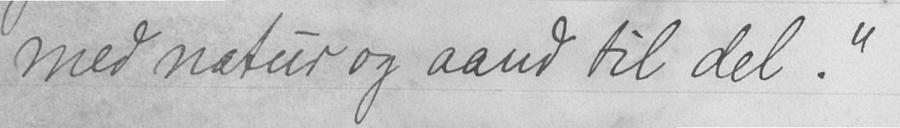med natur og aand til del."
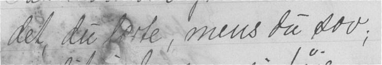det, du lærte, mens du sov;
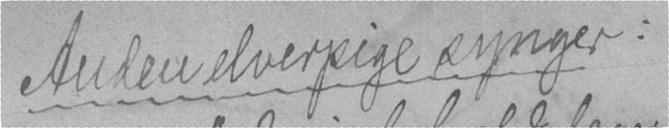Anden elverpige synger:
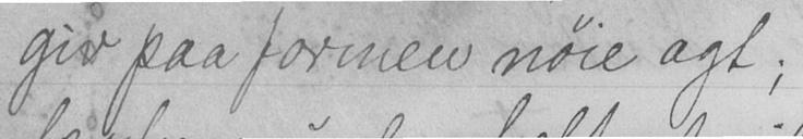giv paa formen nøie agt;
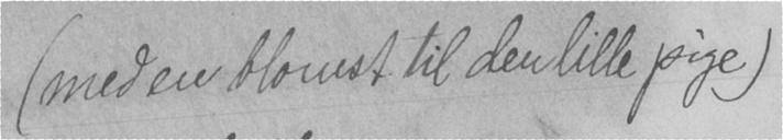(med en blomst til den lille pige)
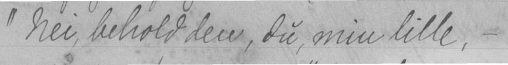"Nei, behold den, du, min lille, -
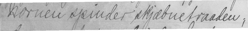"Nornen spinder skjæbnetraaden;
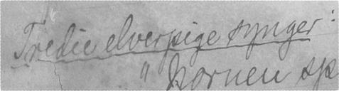Tredie elverpige synger:
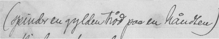(spinder en gylden tråd paa en håndten)
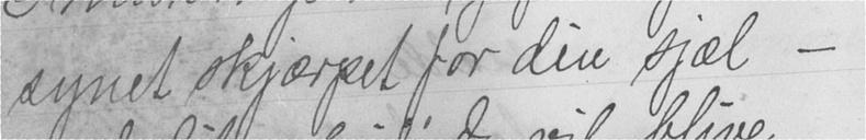synet skjærpet for din sjæl -
